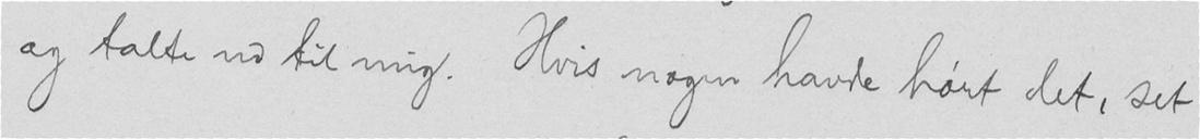og talte ud til mig. Hvis nogen havde hørt det, set
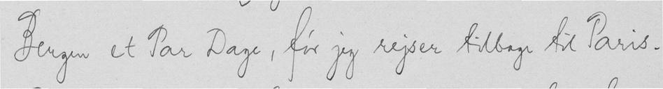Bergen et Par Dage, før jeg rejser tilbage til Paris.
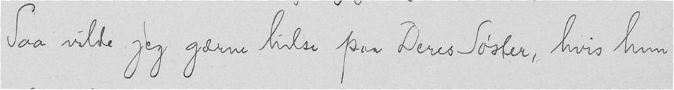Saa vilde jeg gærne hilse paa Deres Søster, hvis hun
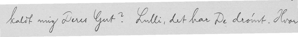kaldt mig Deres Gut? Lulli, det har De drømt. Hvor
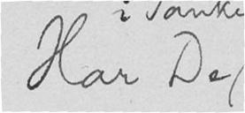Har De
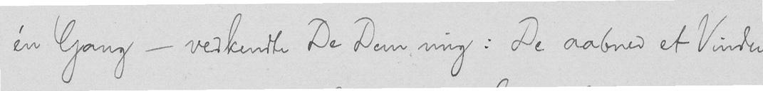én Gang - vedkendte De Dem mig: De aabned et Vindu
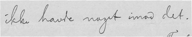ikke havde noget imod det.
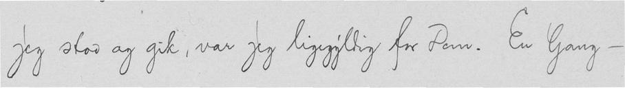jeg stod og gik, var jeg ligegyldig for Dem. En Gang -
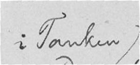i Tanken
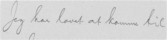Jeg har lovet at komme til
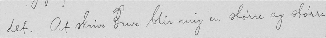det. At skrive Breve blir mig en større og større
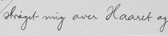strøget mig over Haaret og
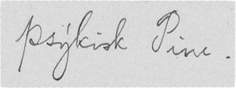psykisk Pine.
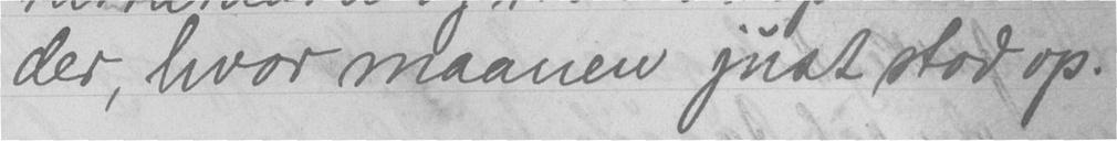der, hvor maanen just stod op.
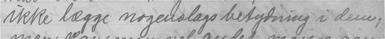ikke lægge nogenslags betydning i dem;
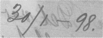30/1- 98.
